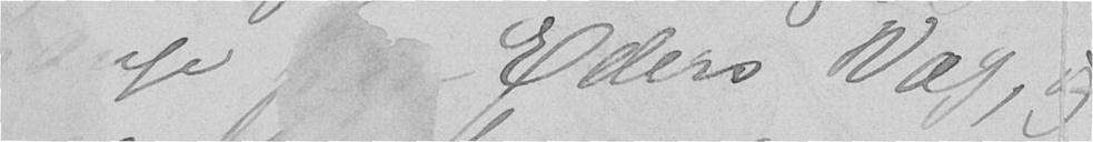ge fod Eders Væg, og
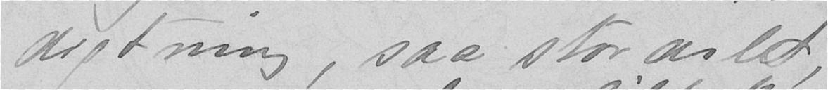digtning, saa storartet,
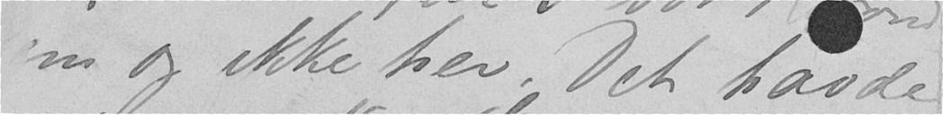og ikke her. Det havde
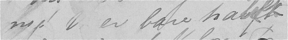mig! Vi er bare halft
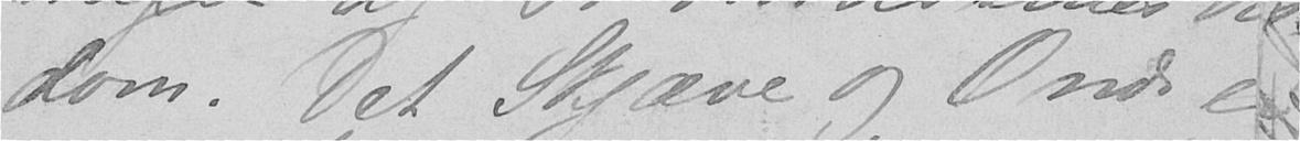dom. Det Skjæve og Onde er
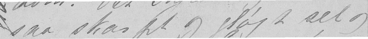saa skarpt og gløgt set og
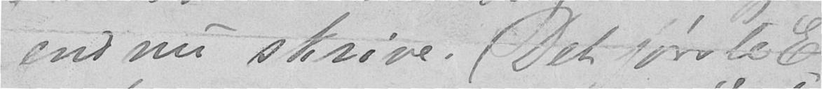endnu skrive. Det første E-
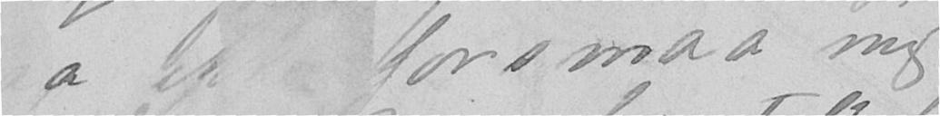a ek forsmaa mig
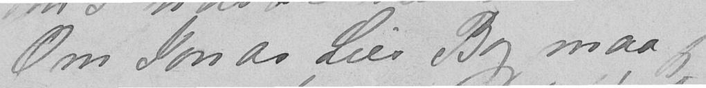Om Jonas Lies Bog maa jeg
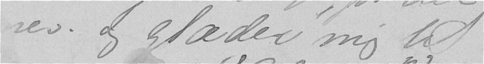rer. Jeg glæder mig til
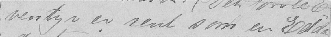ventyr er rent som en Edda-
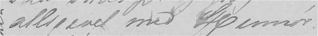alligevel med Humør.
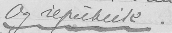Og republik.
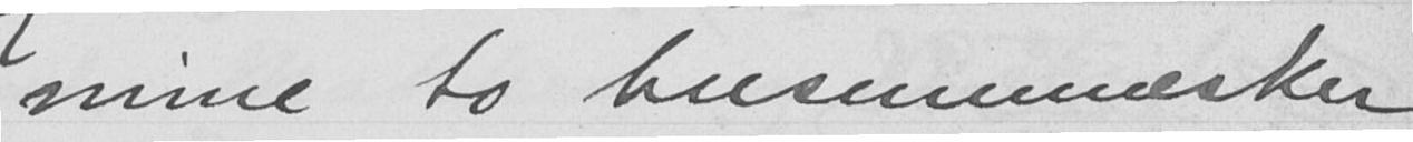mine to husmennesker
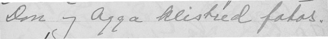Don og Agga klistred fotos.
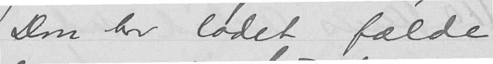Don har ladet fælde
